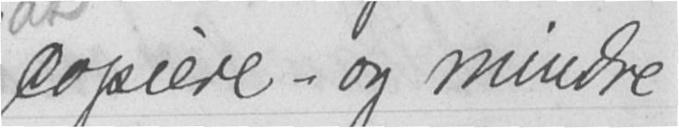copiere - og mindre
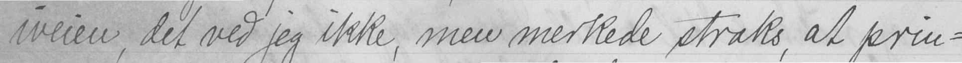iveien, det ved jeg ikke, men merkede straks, at prin-
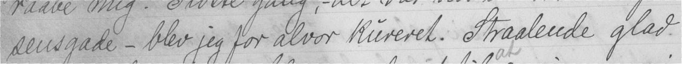sensgade - blev jeg for alvor kureret. Straalende glad
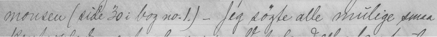monsen (side 30 i bog. no. 1.) - Jeg søgte alle mulige smaa
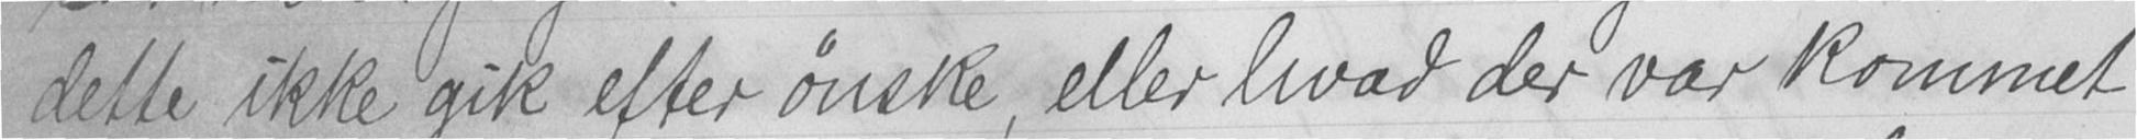dette ikke gik efter ønske, eller hvad der var kommet
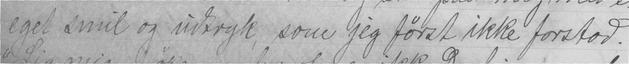eget smil og udtryk, som jeg først ikke forstod.
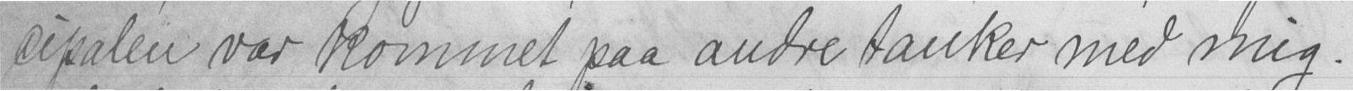cipalen var kommet paa andre tanker med mig.
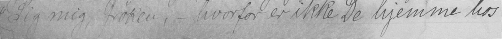"Sig mig, frøken, - hvorfor er ikke De hjemme hos
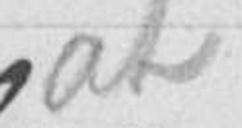at
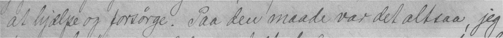at hjælpe og forsørge. Paa den maade var det altsaa, jeg
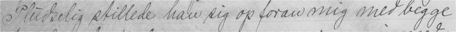Pludselig stillede han sig op foran mig med begge
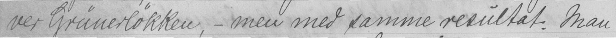ver Grünerløkken, - men med samme resultat. Man
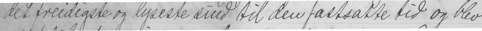det freidigste og lyseste sind til den fastsatte tid og blev
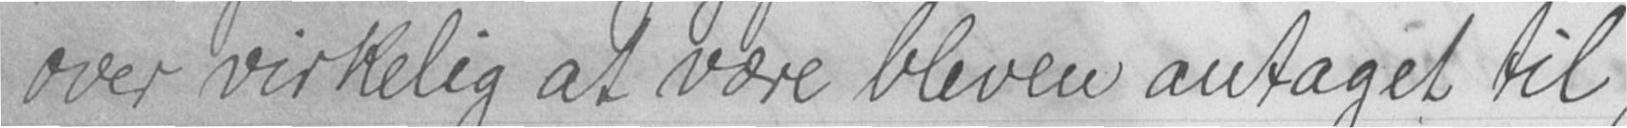over virkelig at være bleven antaget til
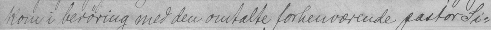kom i berøring med den omtalte forhenværende pastor Si-
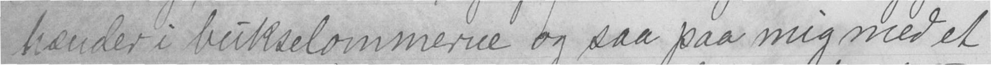hænder i bukselommerne og saa paa mig med et
